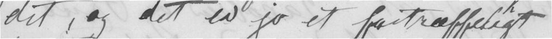det, og det er jo et fortræffeligt
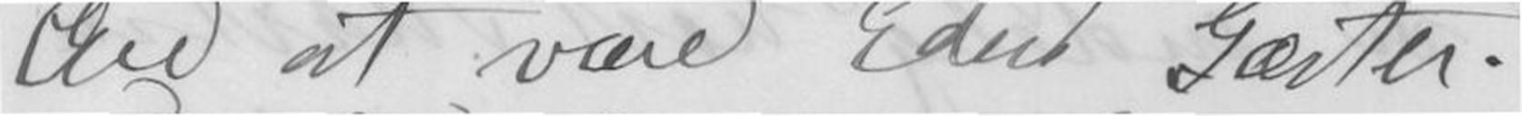Ære at være Eders Gæster.
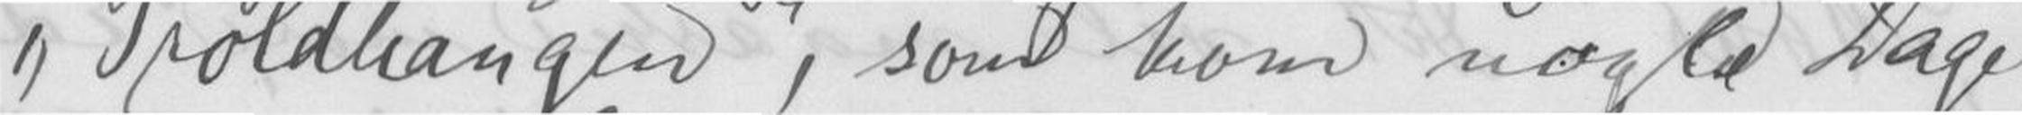Troldhaugen, som kom nogle Dage
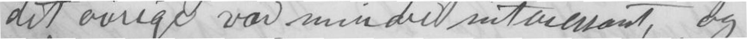det øvrige var mindre interessant, og
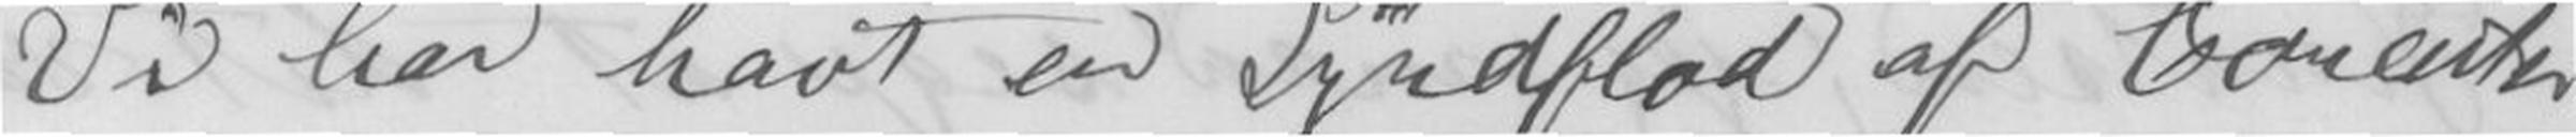Vi har havt en Syndflod af Concerter
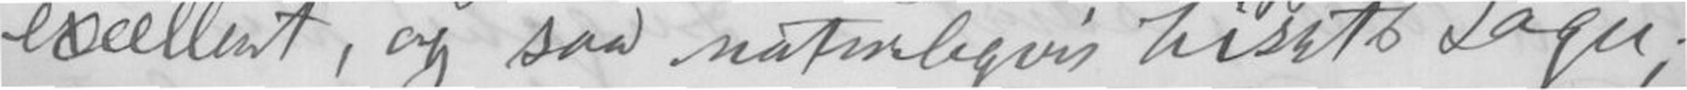excellent, og saa naturligvis Liszts Sager,
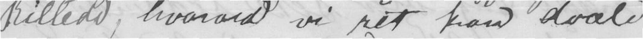Billede, hvorved vi ret kan dvæle
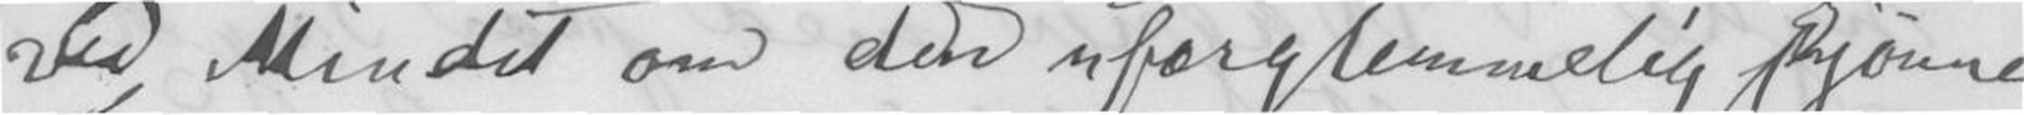ved Mindet om den uforglemmelig skjønne
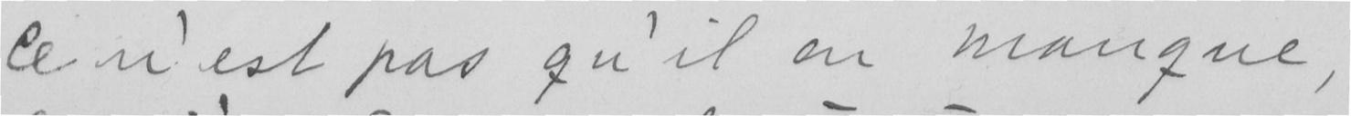le n'est pas qu'il en manqne
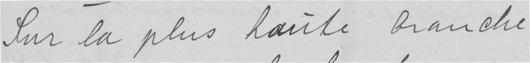Lur la plus hante branche
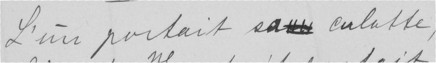L'un portait sa culatte
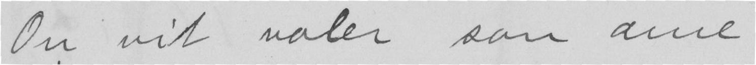On vit valer som ame
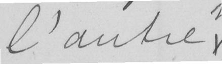l'antre
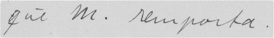que M. remporta.
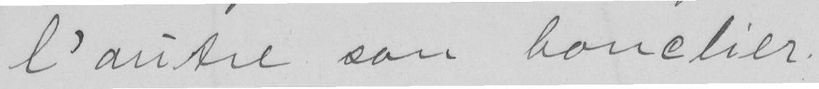l'autre som bonclier.
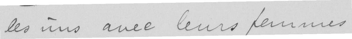les uns avee lenrs femmes
